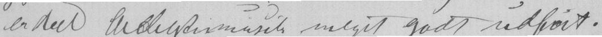en deel Orchestermusik meget godt udført.
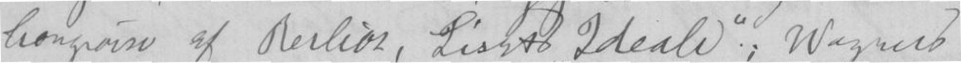hongroise af Berlioz, Liszts Ideale; Wagners
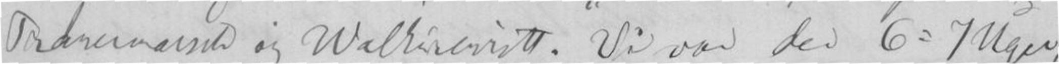Tranemarsch og Walkürenritt. Vi var der 6=7 Uger,
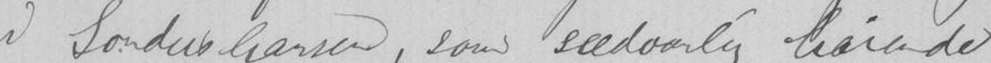jeg i Sondershausen, som sædvanlig hørede
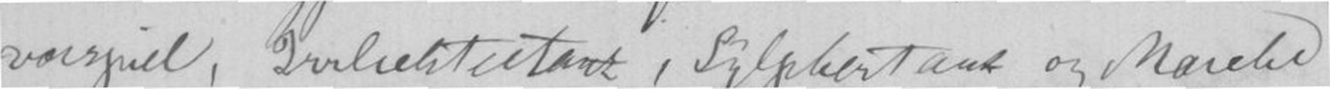vorspiel, Irrlichtertanz. Sylphertanz og Mærche
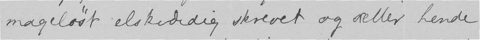mageløst elskværdig skrevet og sætter hende
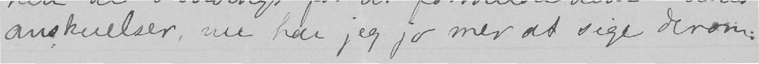anskuelser, nu har jeg jo mer at sige derom.
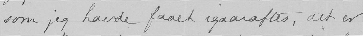som jeg havde faaet igaaraftes, det er
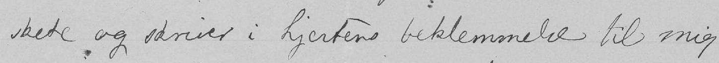skede og skriver i hjertens beklemmelse til mig
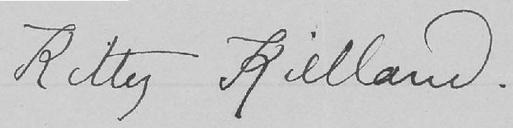Kitty Kielland.
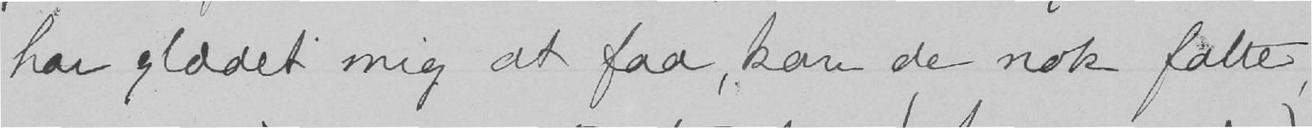har glædet mig at faa, kan de nok fatte,
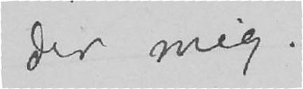der mig.
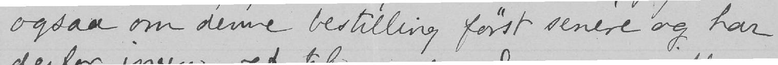ogsaa om denne bestilling først senere og har
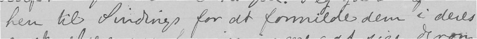hen til Sindings for at formilde dem i deres
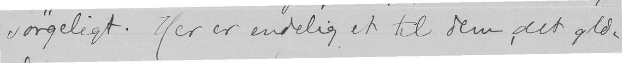sørgeligt. Her er endelig et til dem, det glæ-
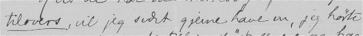tilovers, vil jeg svært gjerne have en, jeg hørte
